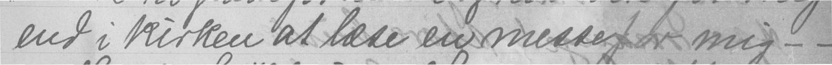end i kirken at læse en messe for mig - -
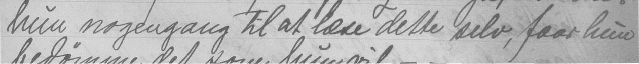hun nogengang til at læse dette selv, faar hun
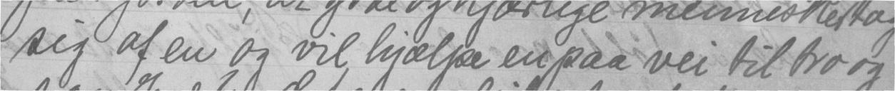sig af en og vil hjælpe en paa vei til tro og
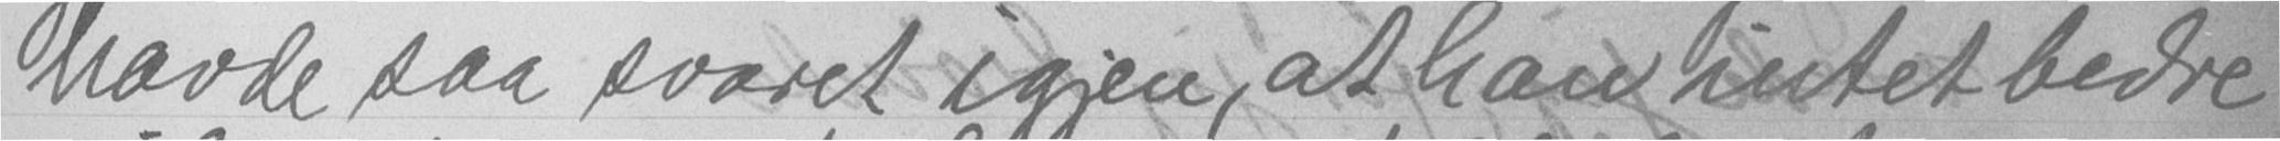havde saa svaret igjen, at han intet bedre
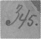345.
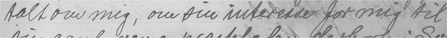talt om mig, om sin interesse for mig til
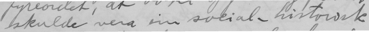skulde vera ein social-historisk
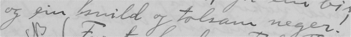og ein snild og tolsom neger!
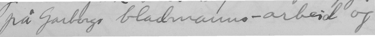på Garborgs bladmanns-arbeid og
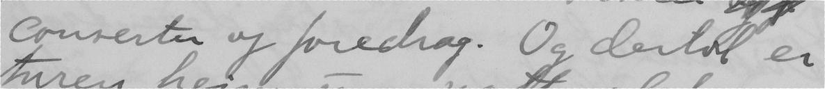Conserter og foredrag. Og dertil er
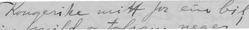Kongerike mitt for ein bil
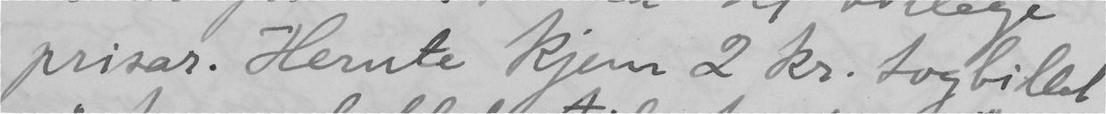prisar. Herute kjem 2 kr. togbillet
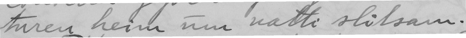turen heim um natti slitsam.
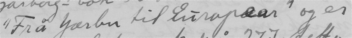"Frå Jærbu til Europear" og er
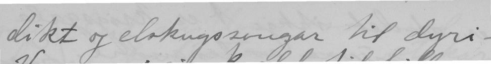dikt og elskugssongar til dyri-
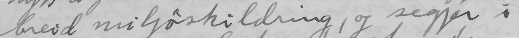breid miljöskildring, og segjer i
